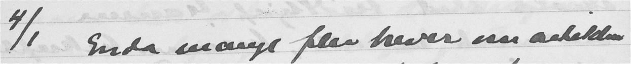4/1 Enda mange fler brever om artiklene
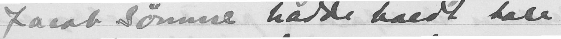Jacob Sømme hadde holdt tale
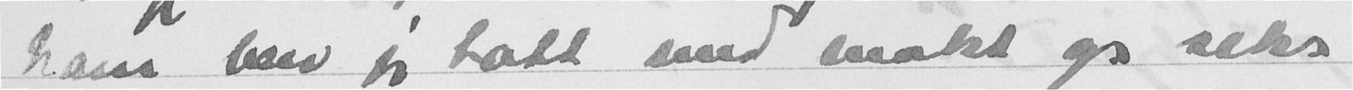ham blev jeg tatt med makt op seks
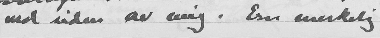ved siden av mig. En merkelig
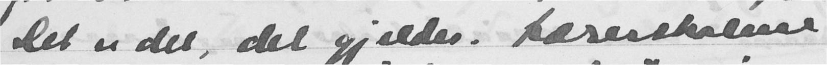Det er det, det gjelder. Lærerskolene
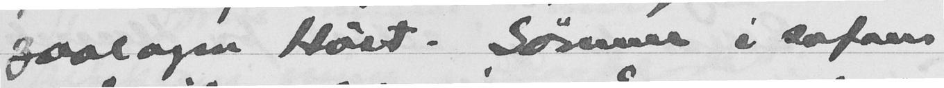geologen Høst. Sønnen i sofaen
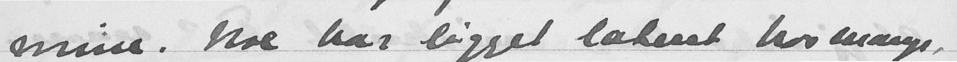mine. Noe har ligget latent hos mange,
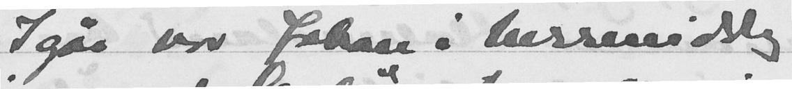Igår var Johan i herremiddag
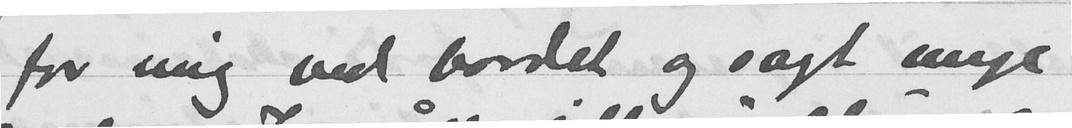for mig ved bordet og sagt mye
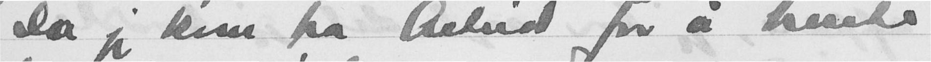Da jeg kom fra Astrid for å hente
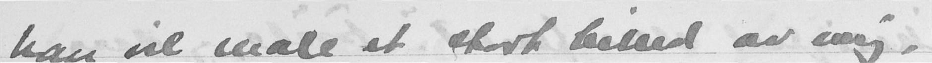han vil male et stort billed av mig,
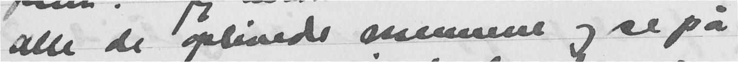alle de oplivede mennene og se på
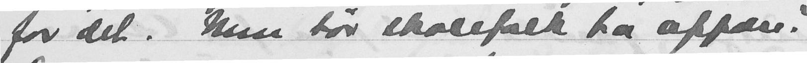for det. Men bør skolefolk ta affære?
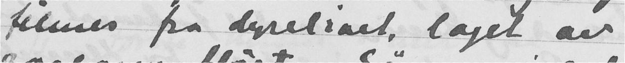filmer fra dyrelivet, laget av
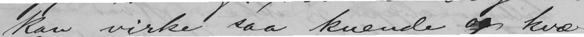kan virke saa kuende og kvæ-
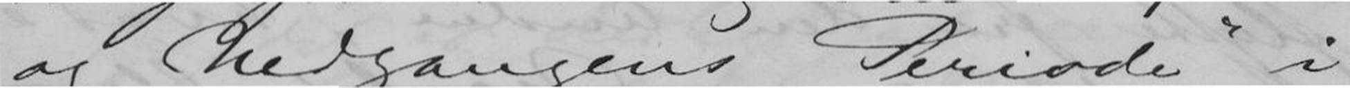og Nedgangens Periode" i
